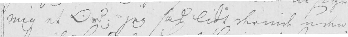mig et Ord; jeg sat lidt derinde uden
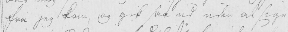fra jeg kom, og gik saa ud uden at sige
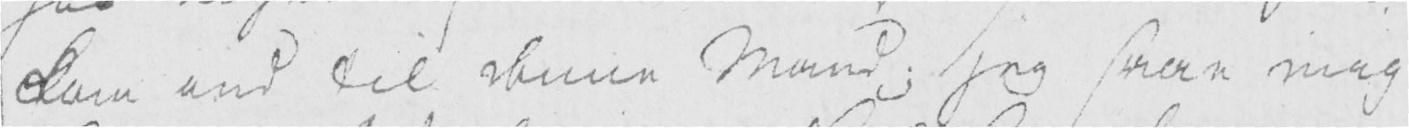kom ind til denne Mand; jeg saae mig
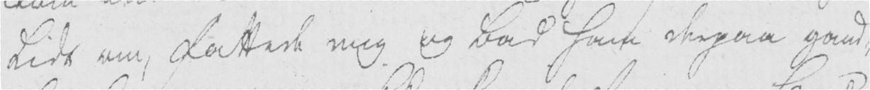lidt om, fattede mig og bad ham derpaa gand-
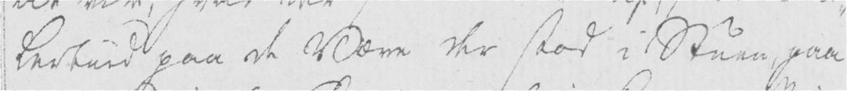Lerkund paa de Væve der stod i Stuen, paa
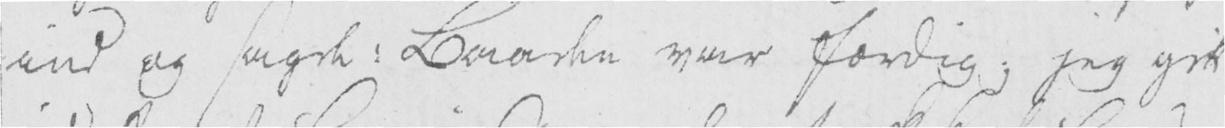ind og sagde: Baaden var færdig; jeg gik
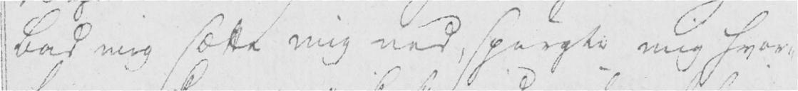bad mig sætte mig ned, spurgte mig hvor-
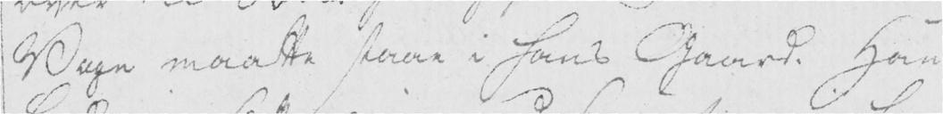Vogn maatte staae i hans Gaard. Han
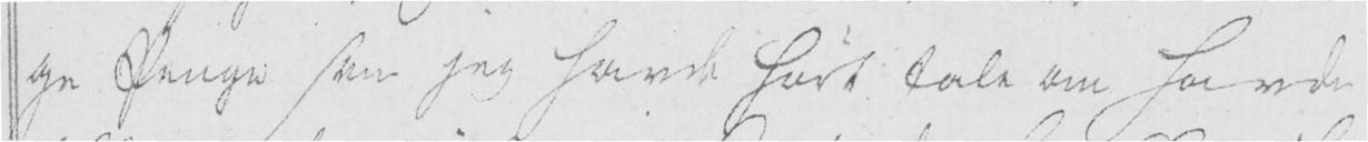ge Penge som jeg havde hørt tale om havde
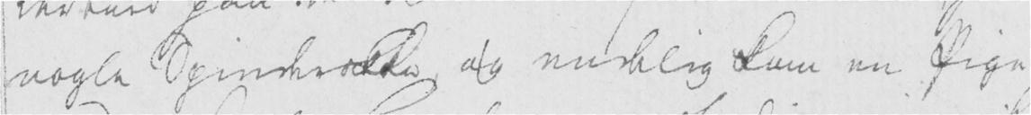nogle Spinderokke, og endelig kom en Pige
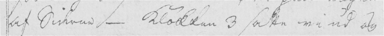af Siderne - Klokken 3 satte vi ud og
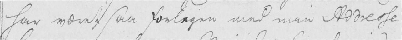har været saa forlegen med min Addresse
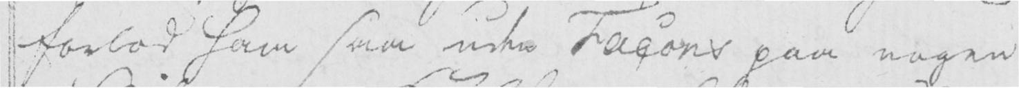forlod ham saa uden Facons paa nogen
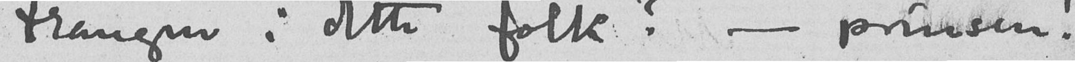trangen i dette folk? - prinsen!
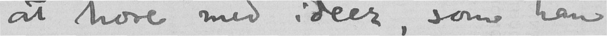at hore med ideer, som han
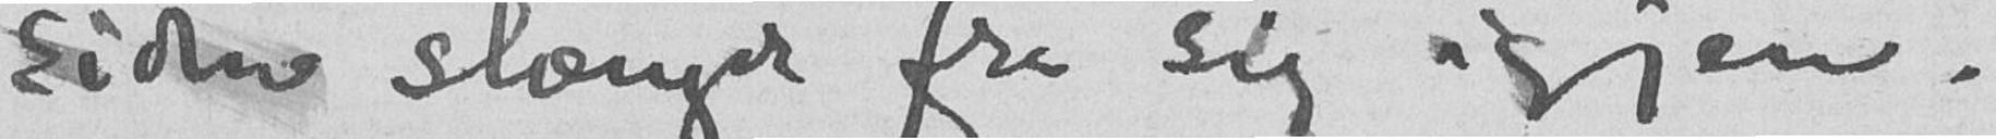siden slænger fra sig igjen.
